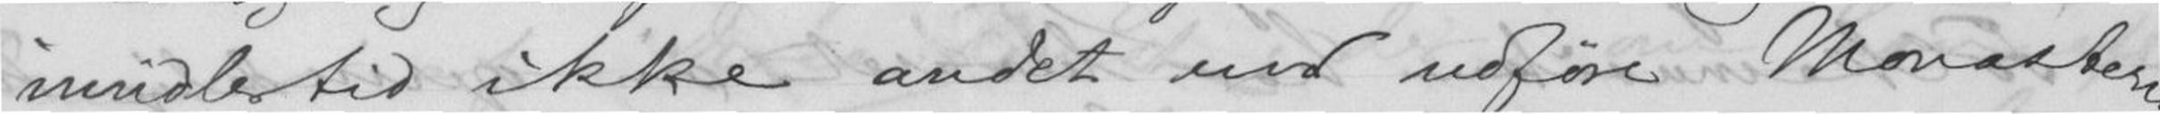imidlertid ikke andet end udføre Monasterios
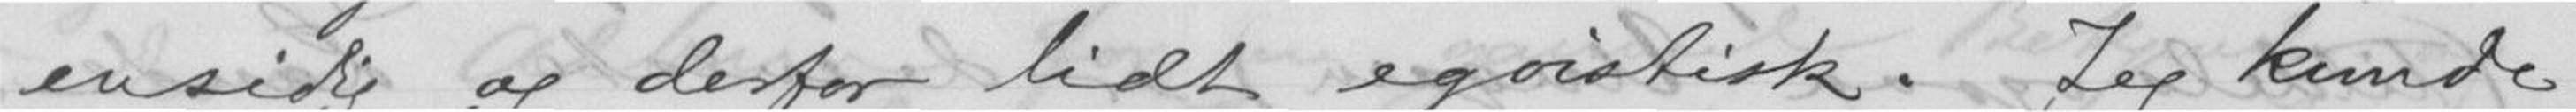ensidig og derfor lidt egoistisk. Jeg kunde
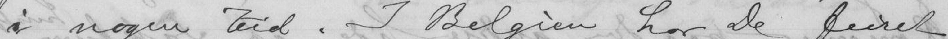i nogen Tid. I Belgien har De feiret
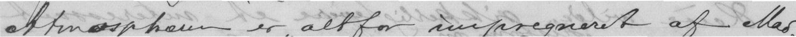Atmosphæren er altfor impregneret af Mad-
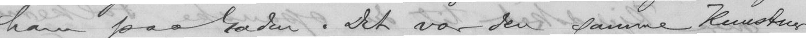han paa Gaden. Det var den samme Kunstner,
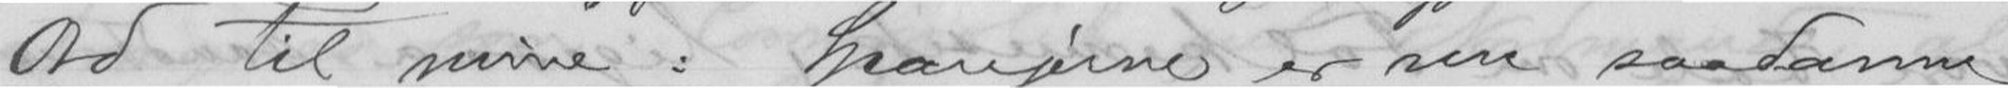Ord til mine: Spanjerne er nu saadanne
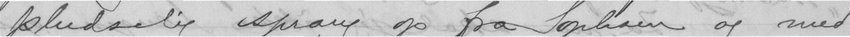pludselig sprang op fra Sophaen og med
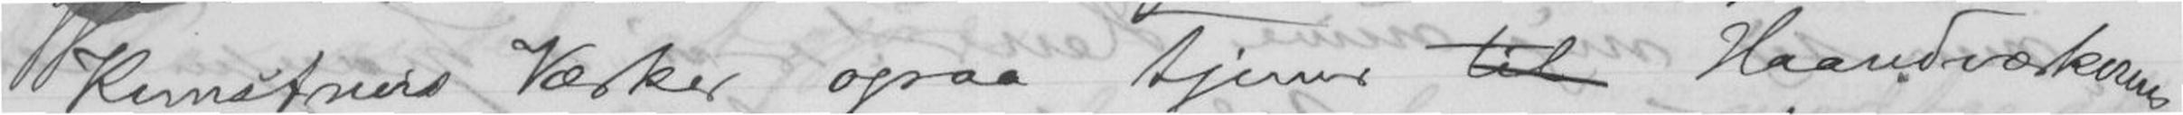Kunstners Værker ogsaa tjener til Haandværkernes
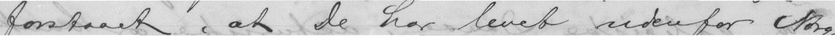forstaaet, at De har levet udenfor Norge
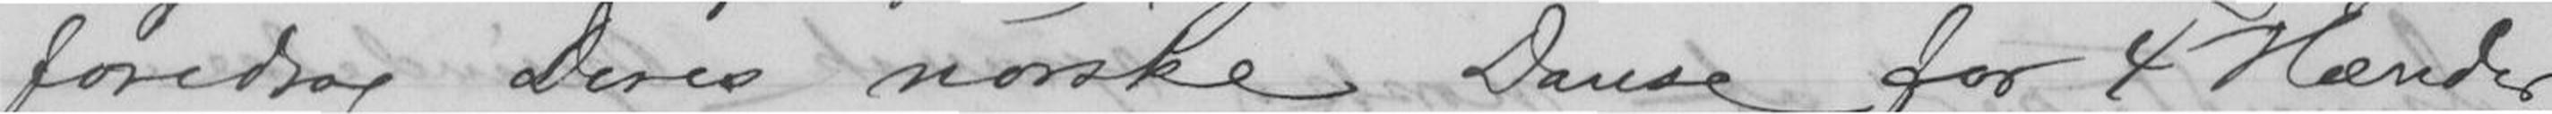foredrog Deres norske Danse for 4 Hænder
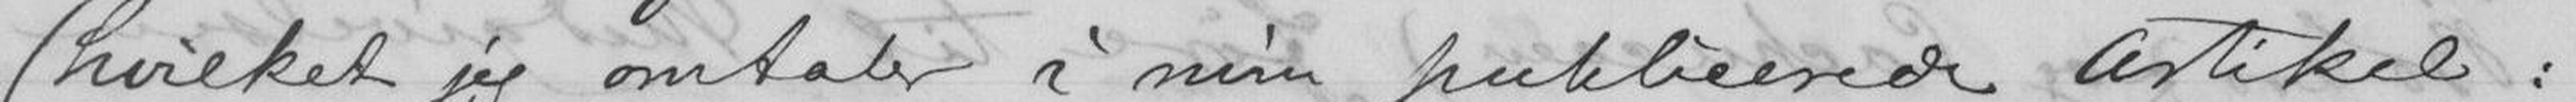(hvilket jeg omtaler i min publicerede Artikel:
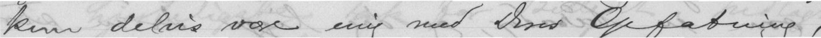kun delvis være enig med Deres Opfatning,
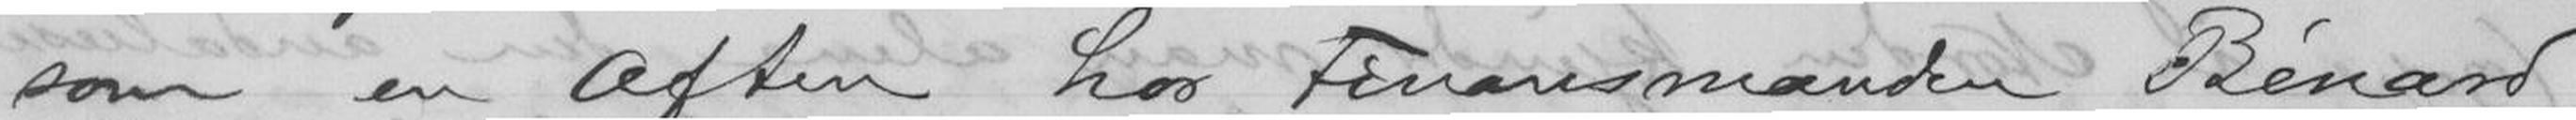som en Aften hos Finansmanden Bènard
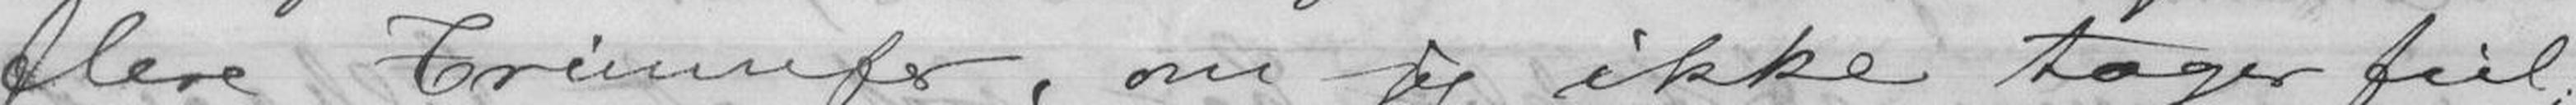flere Triumfer, om jeg ikke tager feil;
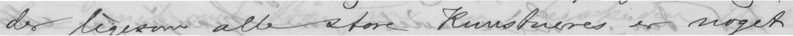der ligesom alle store Kunstneres er noget
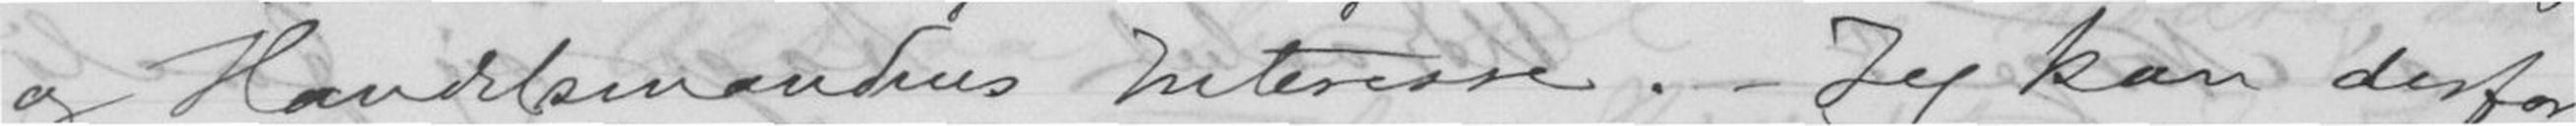og Handelsmandens Interesse. - Jeg kan derfor
